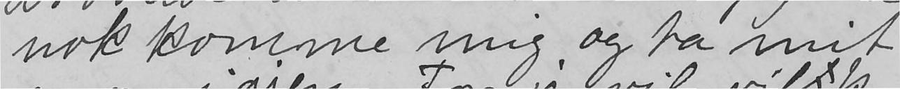nok komme mig og ta mit
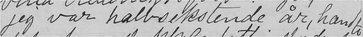jeg var halvsekstende år, hændte
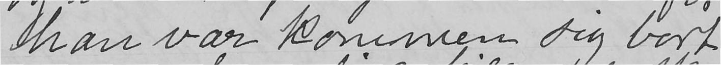han var kommen sig bort
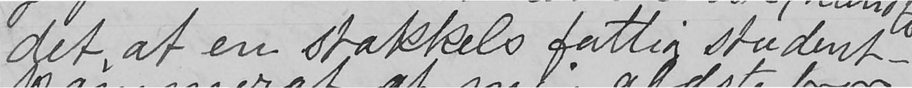det, at en stakkels fattig student-
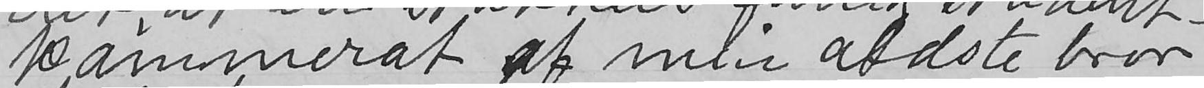kammerat af min ældste bror
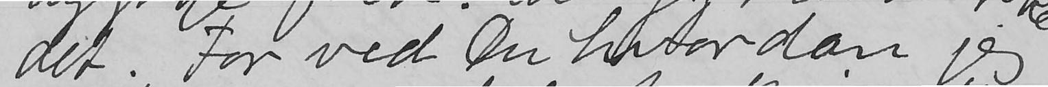det. For ved Du hvordan jeg
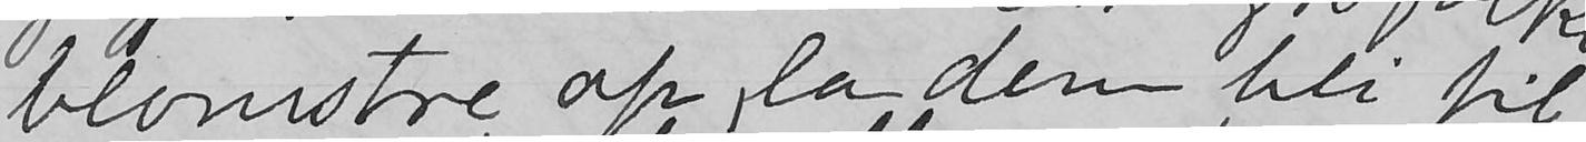blomstre op, la dem bli til
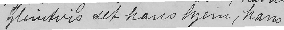glimtvis set hans hjem, hans
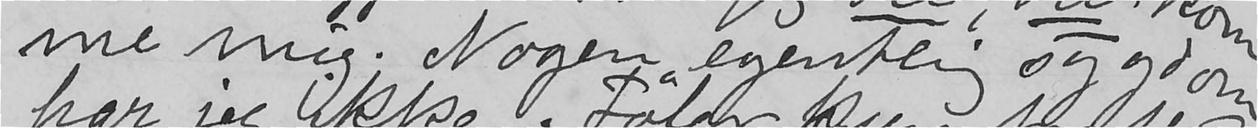me mig. Nogen egentlig sygdom
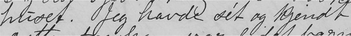huset. Jeg havde sét og kjendt
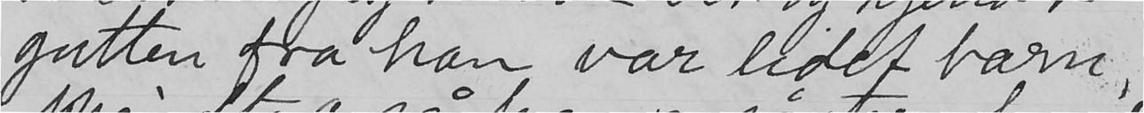gutten fra han var lidet barn,
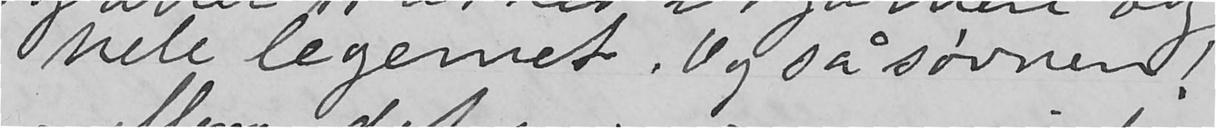hele legemet. Og så søvnen!
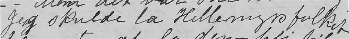Jeg skulde la Hellemyrsfolket
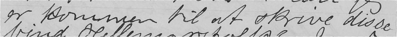er kommen til at skrive disse
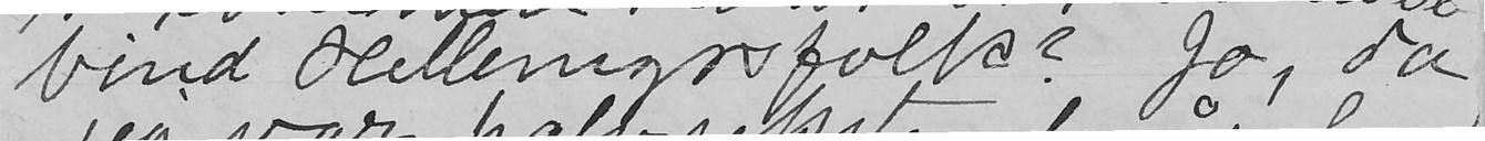bind Hellemyrsfolk? Jo, da
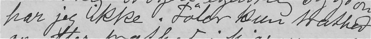har jeg ikke. Føler kun træthed
